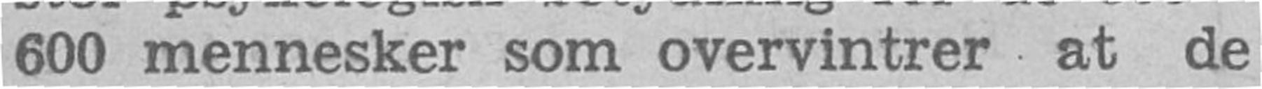600 mennesker som overvintrer at de
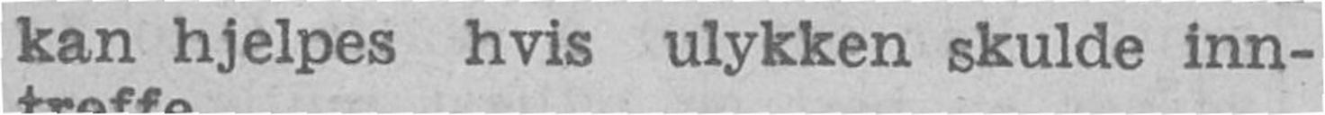kan hjelpes hvis ulykken skulde inn-
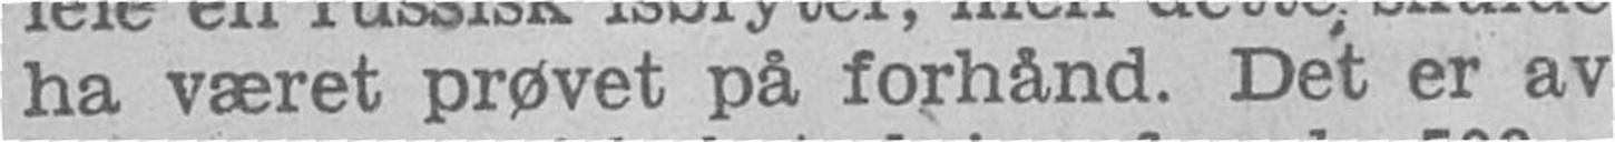ha været prøvet på forhänd. Det er av
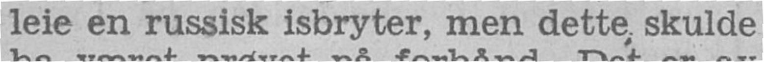leie en russisk isbryter, men dette skulde
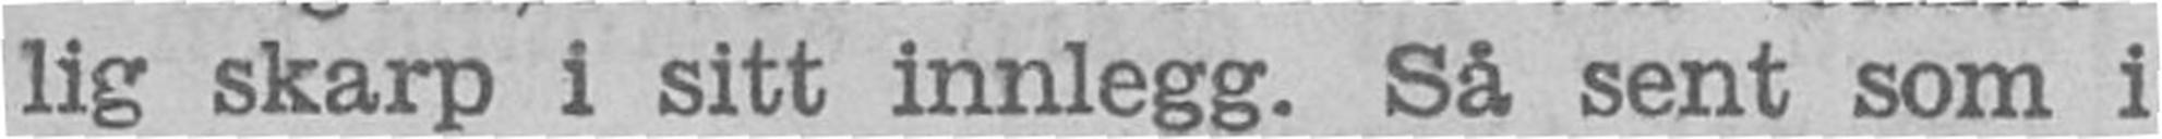lig skarp i sitt innlegg. Så sent som i
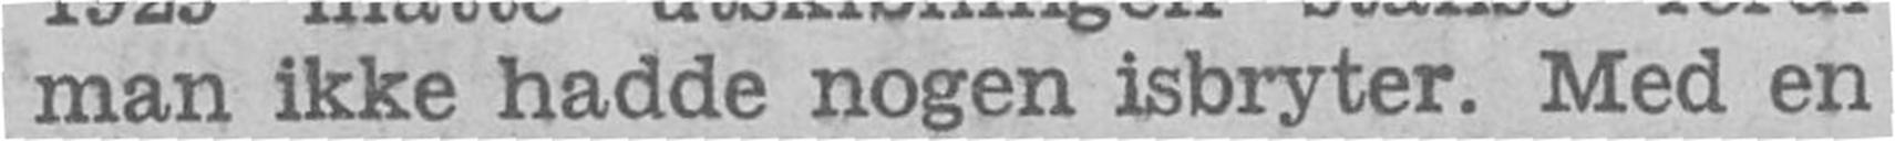man ikke hadde nogen isbryter. Med en
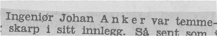Ingeniør Johan Anker var temme-
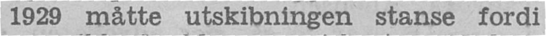1929 måtte utskibningen stanse fordi
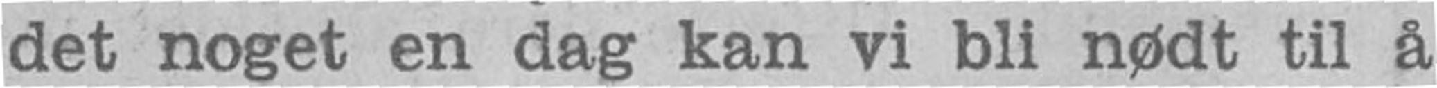det noget en dag kan vi bli nødt til å
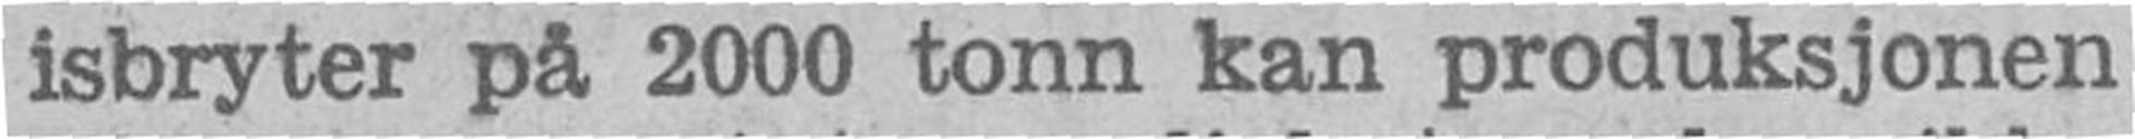isbryter på 2000 tonn kan produksjonen
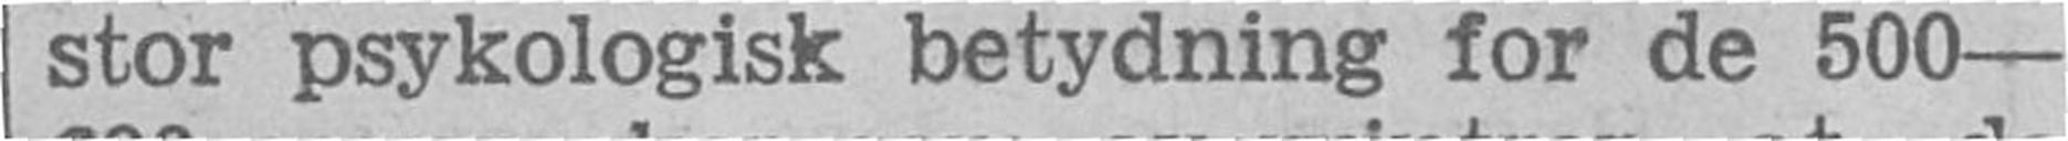stor psykologisk betydning for de 500 -
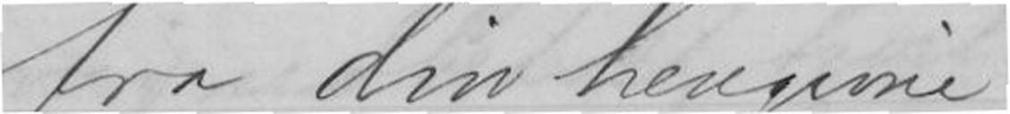fra din hengivne
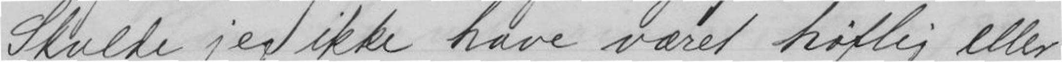Skulde jeg ikke have været høflig eller
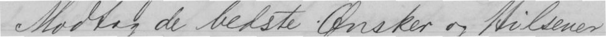Modtag de bedste Ønsker og Hilsener
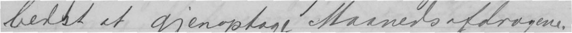bedst at gjenoptage Maaneds afdragene.
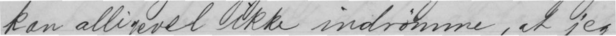kan alligevel ikke indrømme, at jeg
